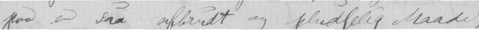paa en saa afbrudt og pludselig Maade,
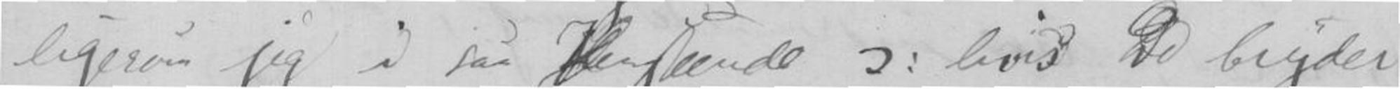ligesom jeg i saa Henseende ): hvis de bryder
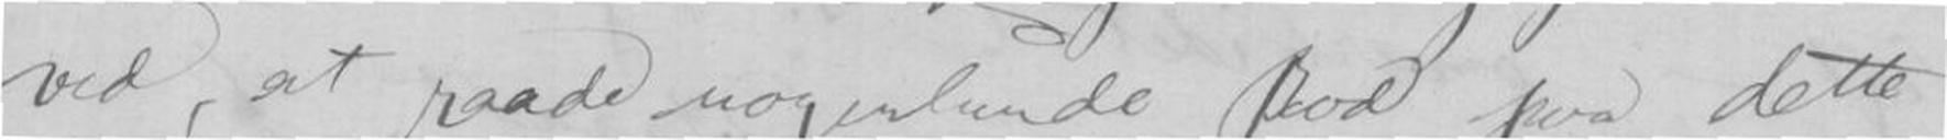ved, at raade nogenlunde Bod paa dette
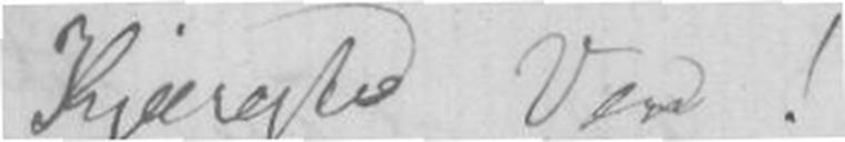Kjæreste Ven!
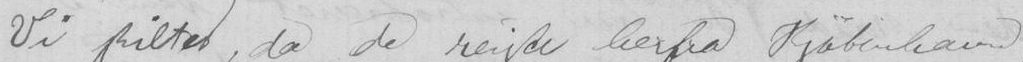Vi skiltes, da De reiste herfra Kjøbenhavn,
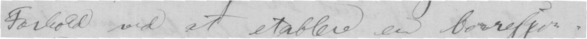Forhold ved at etablere en Correspon-
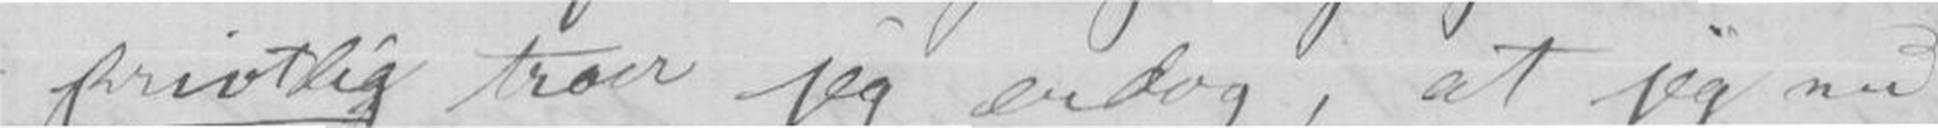skrivtlig tror jeg ændog, at jeg nu
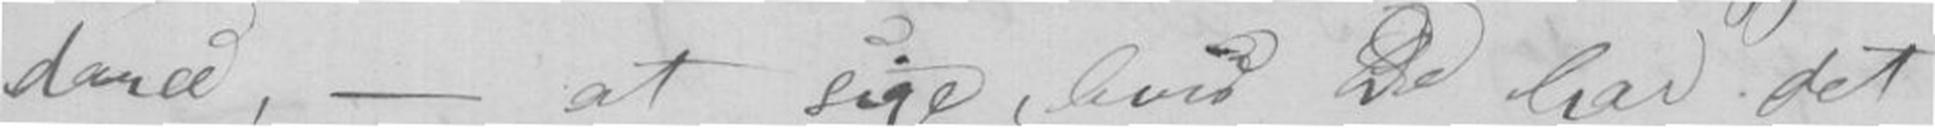dance, - at sige, hvis De har det
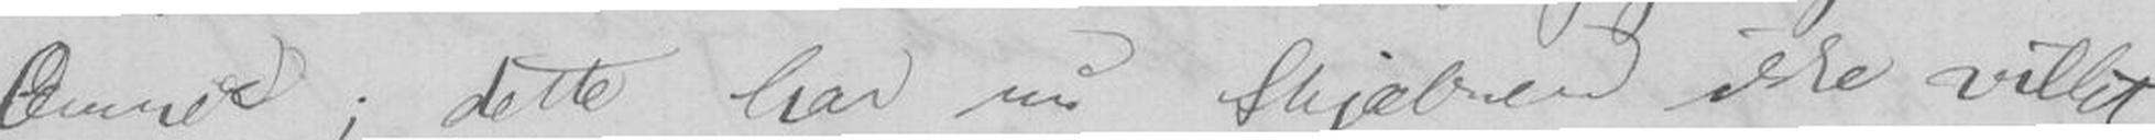Concert; dette har nu Skjæbnen ikke villet
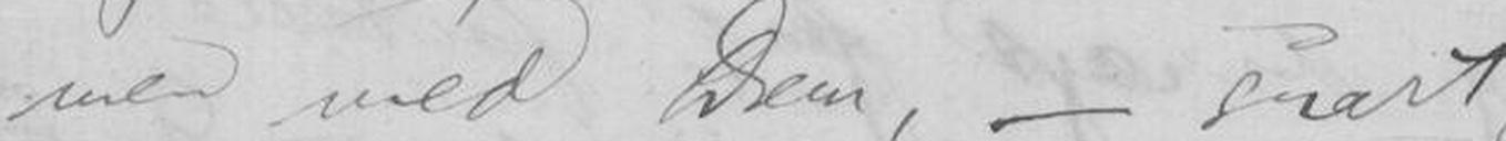men med Dem, - snart
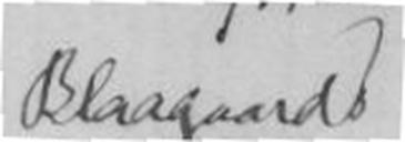Blaagaard
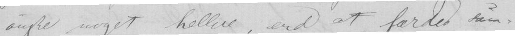ønske noget hellere, end at færdes sam-
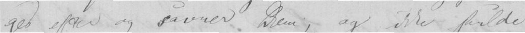ges efter og savner Dem, og ikke skulde
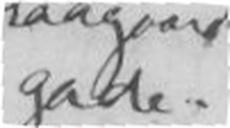gade.
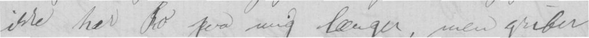ikke har Ro paa mig længer, men griber
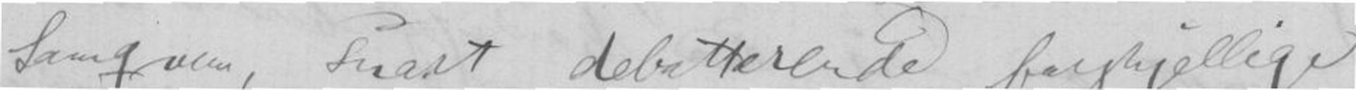Samqvem, snart debatterende forskjellige
